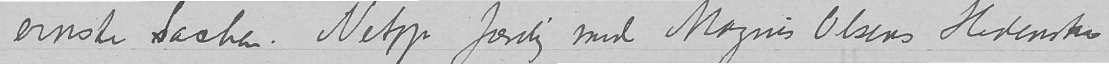ernste Sachen Netop fæerdig med Magnus Olsens Hedenske
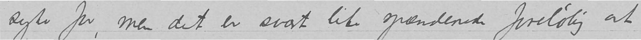syte for, men det er svært lite spændende foreløbig at
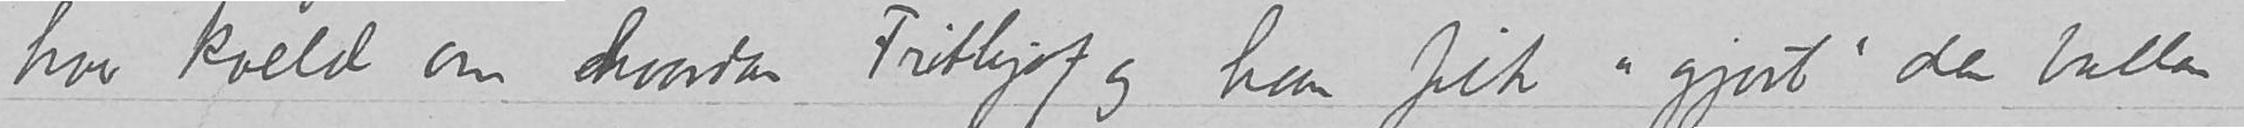hver kveld om hvordan Frithjof og han fik «gjort» den ballern
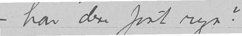har dere faat regn?
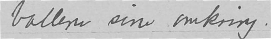ballene sine omkring.
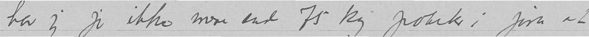Foreløbig har jeg jo ikke mere end 75 kg poteter i jora at
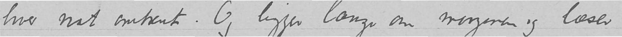hver nat omtrent. Og ligger længe om morgenen og læser
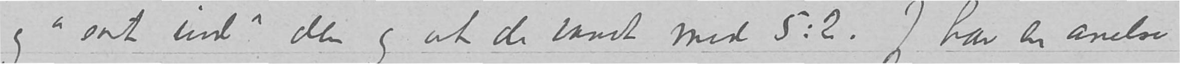og «sat ind» den og at de vandt med 5:2. Jeg har en anelse
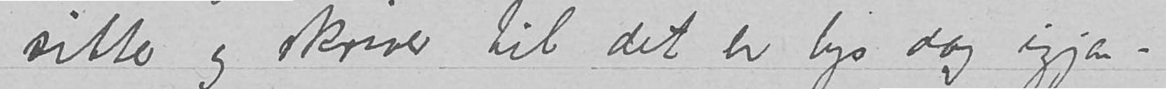Jeg sitter og skriver til det er lys dag igjen -
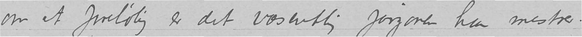om at foreløbig er det væsentlig jargonen han mestrer.
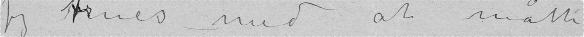jeg trues med at måtte
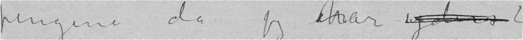pengene da jeg erhar yderst
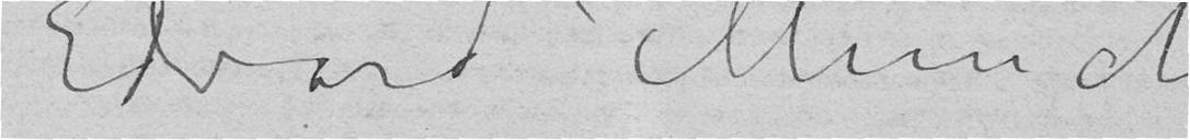Edvard Munch
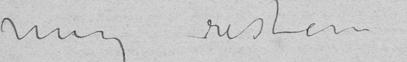mig resten –
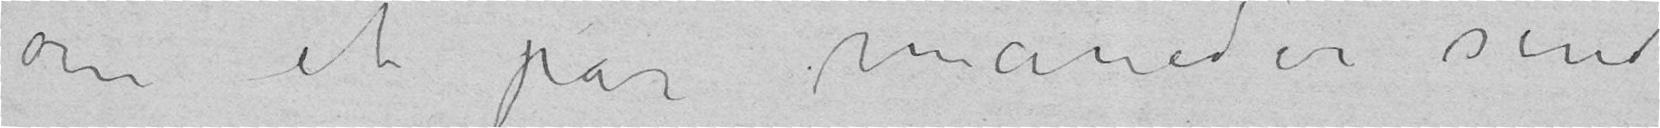om et par måneder sende
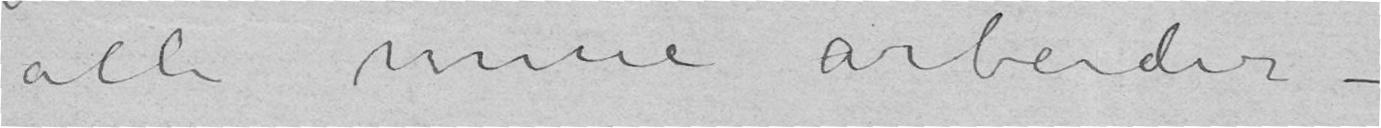alle mine arbeider –
